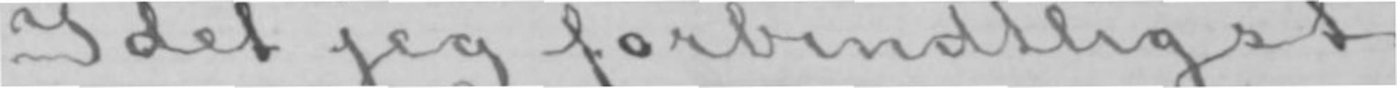Idet jeg forbindtligst
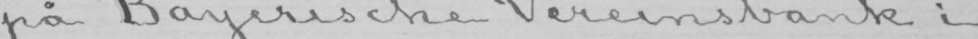på Bayerische Vereinsbank i
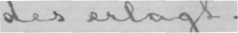des erlagt.
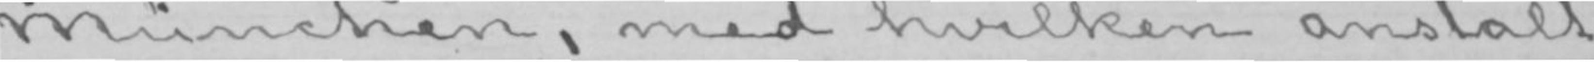München, med hvilken anstalt
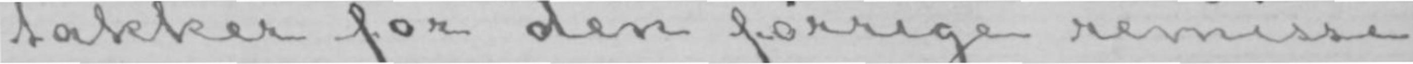takker for den forrige remisse
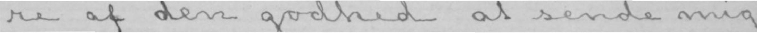re af den godhed at sende mig
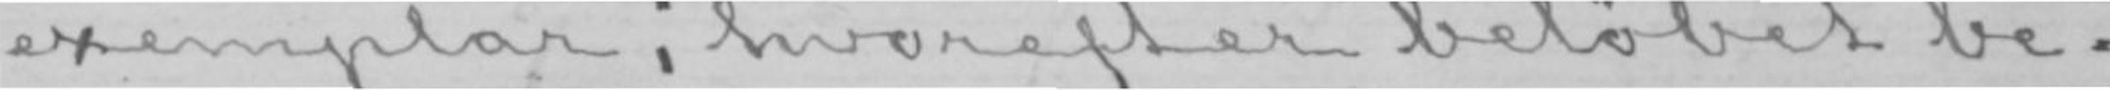exemplar, hvorefter beløbet be-
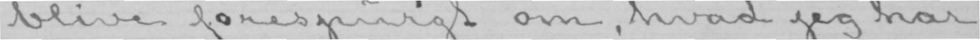blive forespurgt om, hvad jeg har
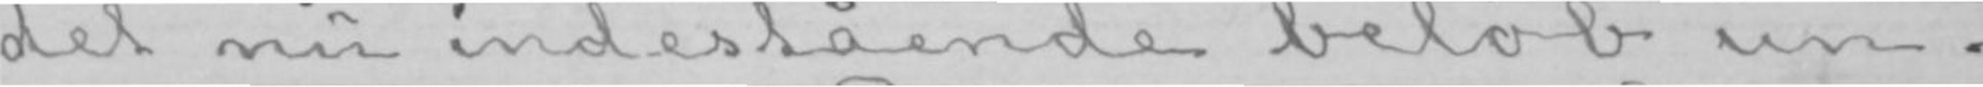det nu indestående beløb un-
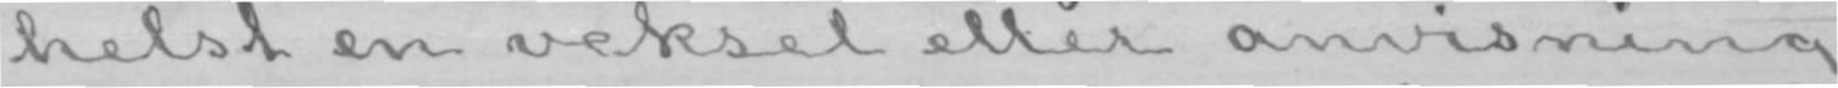helst en veksel eller anvisning
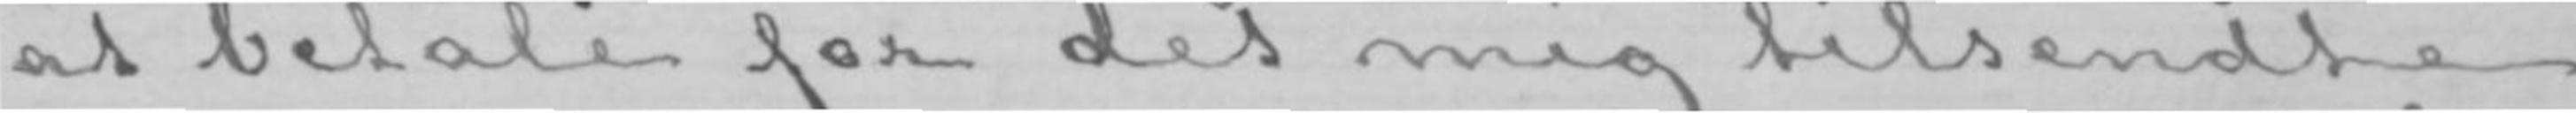at betale for det mig tilsendte
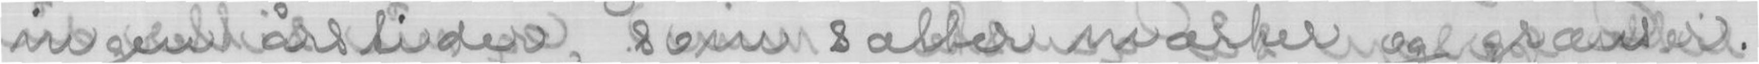ingen årstider, som sætter mærker og grænser.
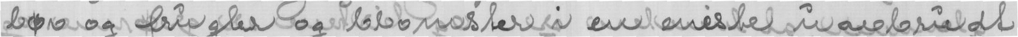løv og frugter og blomster i en eneste uavbrudt
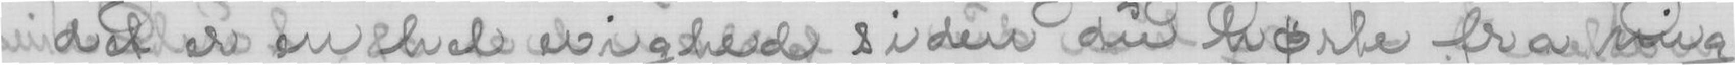det er en hel evighed siden du hørte fra mig,
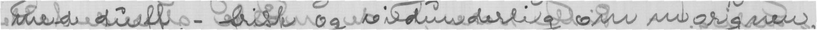med duft, - frisk og vidunderlig om morgen,
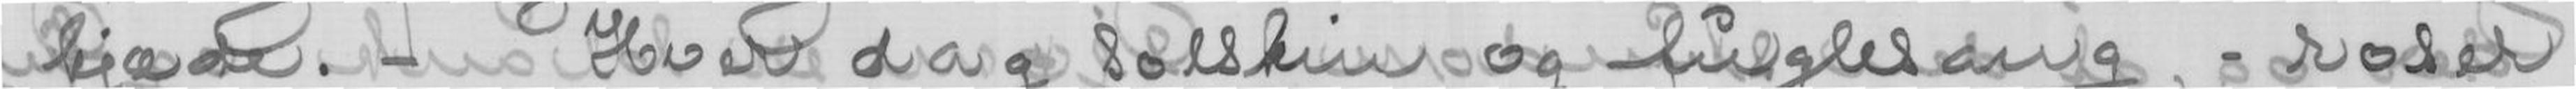kjæde. - Hver dag solskin og fuglesang, - roser
